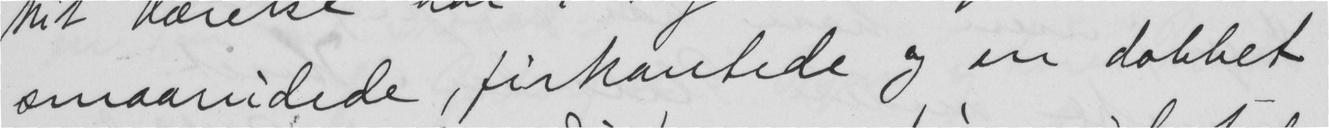smaarudede, firkantede og en dobbet
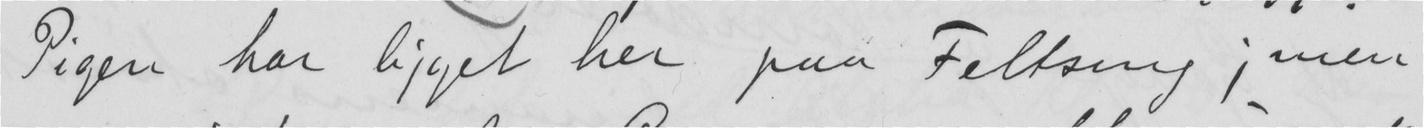Pigen har ligget her paa Feltseng; men
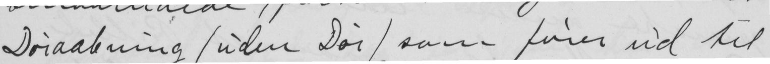Døraabning (uden Dør) som fører ud til
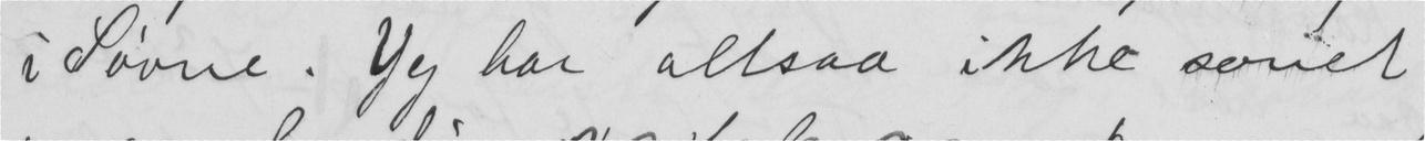i Søvne. Jeg har altsaa ikke sovet
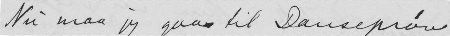Nu maa jeg gaa til Danseprøve
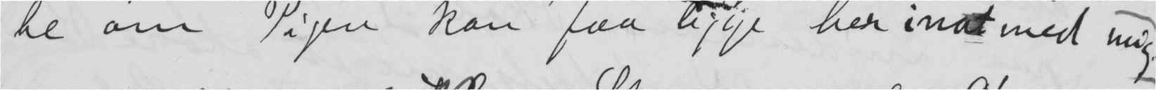be om Pigen kan faa ligge her inat med mig.
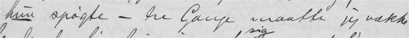hun spøgte - tre Gange maatte jeg vække
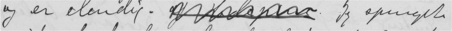og er elendig.  Jeg spurgete
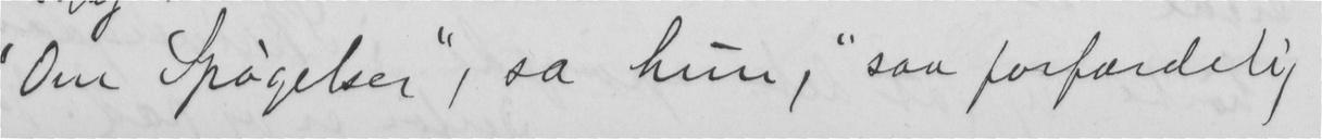"Om Spøgelser", sa hun, "saa forfærdelig
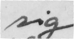sig
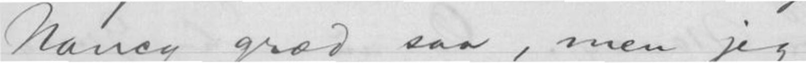Nancy græd saa, men jeg
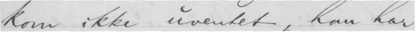kom ikke uventet, han har
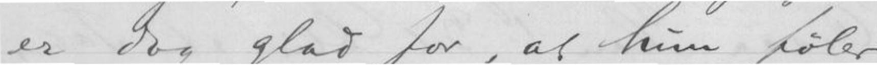er dog glad for, at hun føler
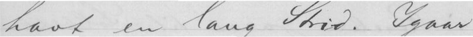havt en lang Strid. Igaar
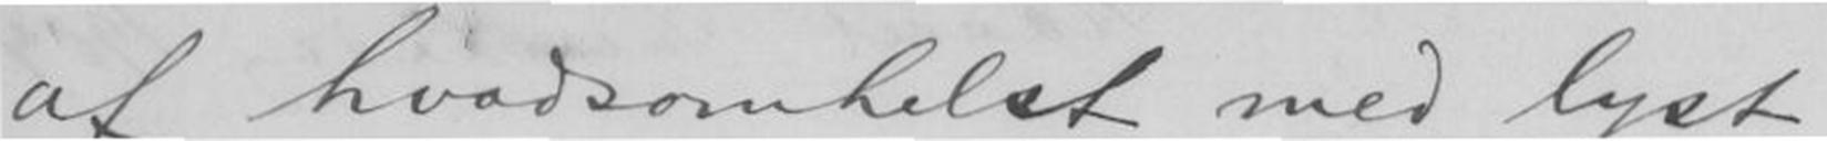af hvadsomhelst med lyst
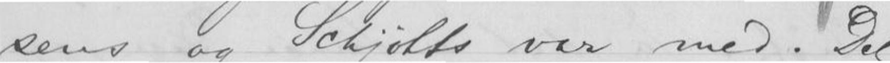sens og Schjøtts var med. Det
